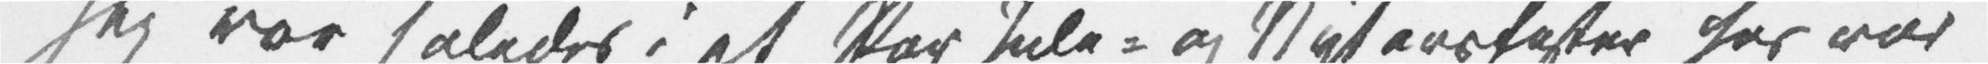Jeg var således i et Par Jule- og Nytårsfester for vor
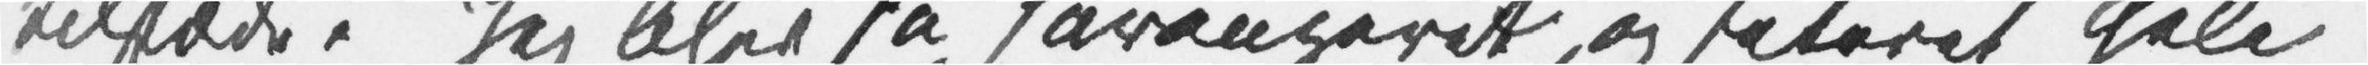tilstæde. Jeg blev så harangeret og feteret hele
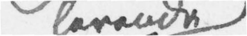levende
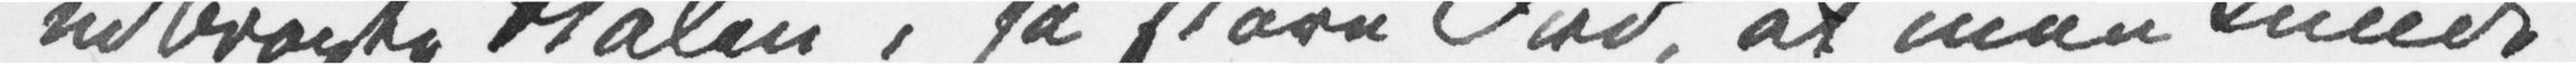udbragte Skålen i så store Ord, at man kunde
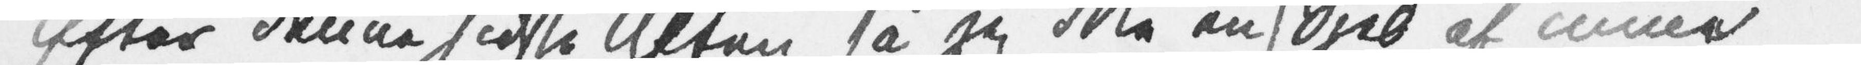Efter denne sidste Aften så jeg ikke en Sjel af mine
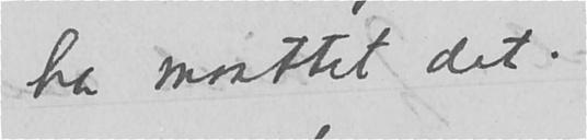ha maattet det.
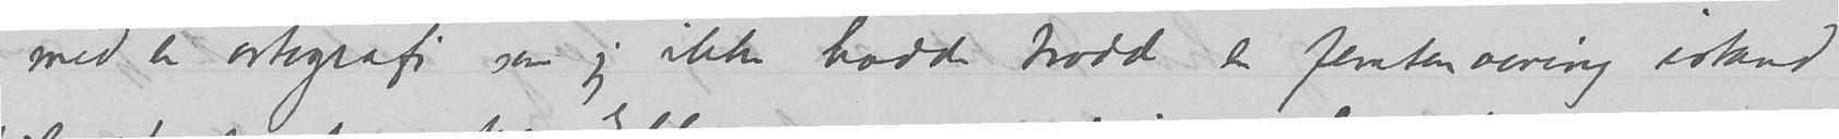med en ortografi som jeg ikke hadde trodd en femtenåring istand
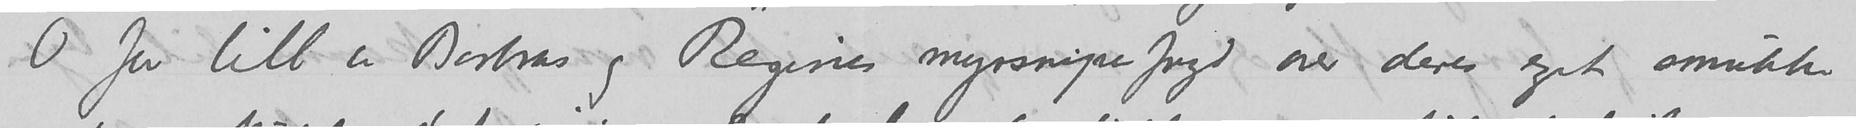O for litt av Barbras og Regines myrsnipe fryd over deres eget smukke
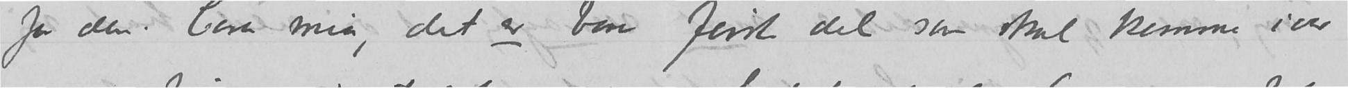for den. Cora mia, det er bare første del som skal komme iaar
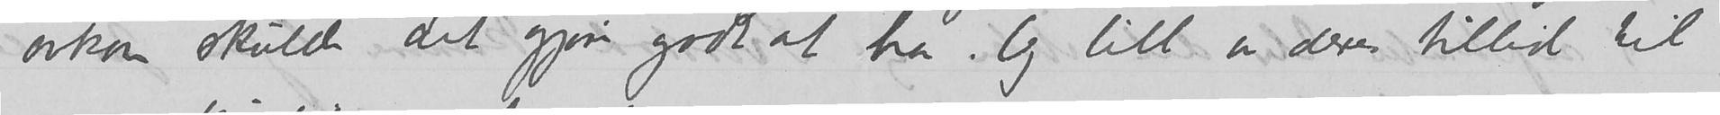avkom skulde det gjøre godt at ha. Og litt av deres tillid til
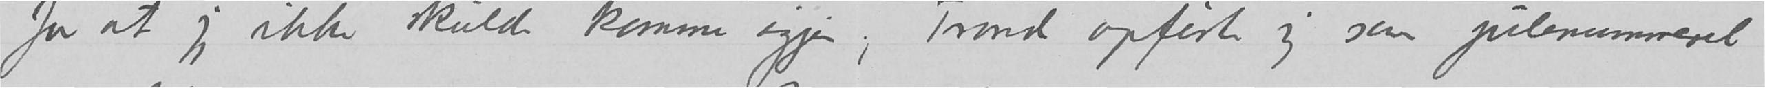for at jeg ikke skulde komme igjen; Trond oppførte sig som julenummeret
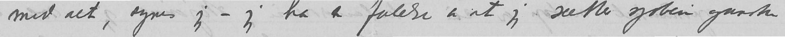med alt, synes jeg – jeg har er følelse av at jeg sætter sjøbein ganske
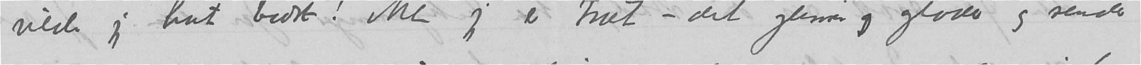vilde jeg hat bedstdst! Men jeg er træt – det glinser/glimrer og gløder og render
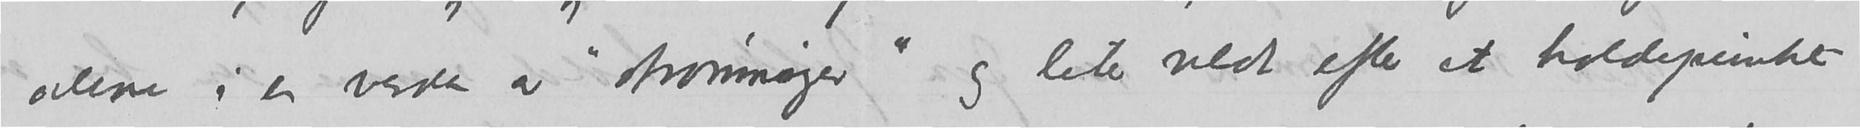alene i en verden av «strømninger» og leter vildt efter et holdepunkt
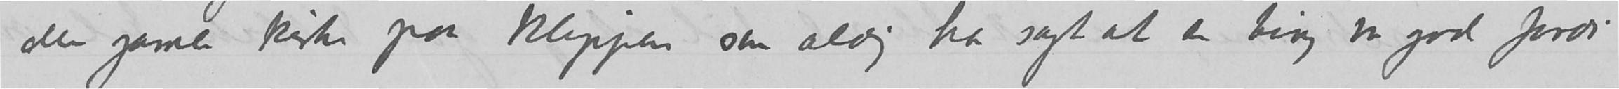den gamle kirke paa klippen som aldrig har sagt at en ting var god fordi
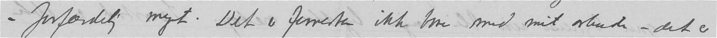– forfærdelig meget. Det er forresten ikke bare med mit arbeide – det er
